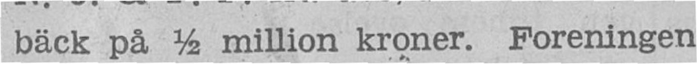bäck på 1/2 million kroner. Foreningen
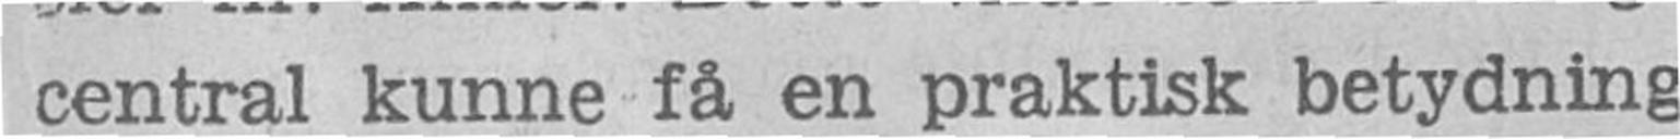central kunne få en praktisk betydning
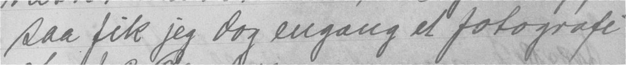saa fik jeg dog engang et fotografi
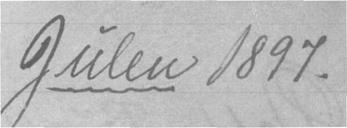Julen 1897.
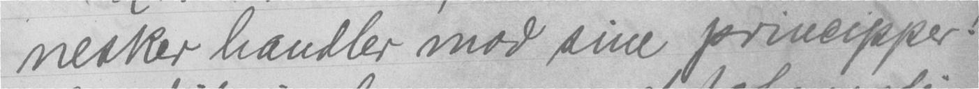nesker handler mod sine principper!
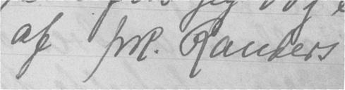af frk. Randers - -
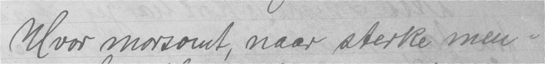Hvor morsomt, naar sterke, men-
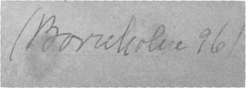(Bornholm 96)
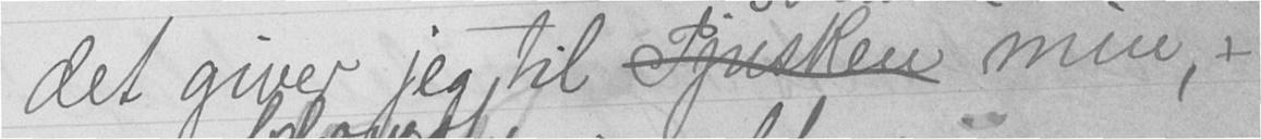det giver jeg til Pjusken vennen min, -
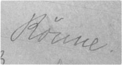Rønne
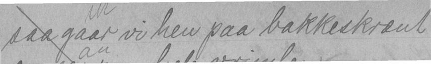saa gaar vi hen paa bakkeskrænt
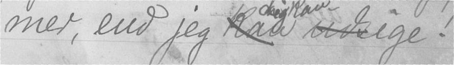mer, end jeg kan sige!
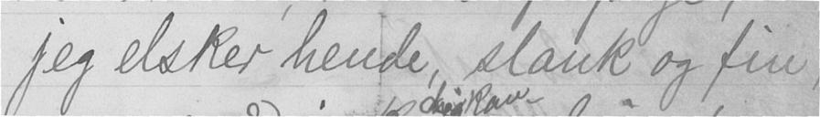jeg elsker hende, slank og fin,
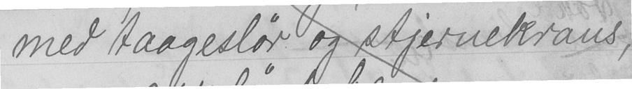med taageslør og stjernekrans,
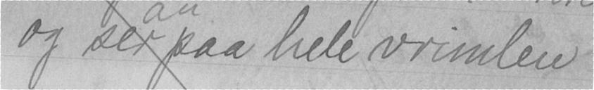og ser paa hele vrimlen
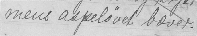mens aspeløvet bæver.
